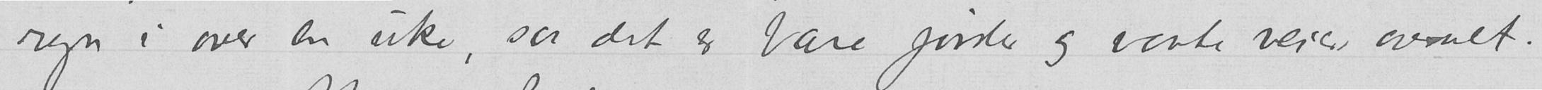regn i over en uke, saa det er bare jorder og vaate veier overalt.
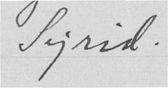Sigrid.
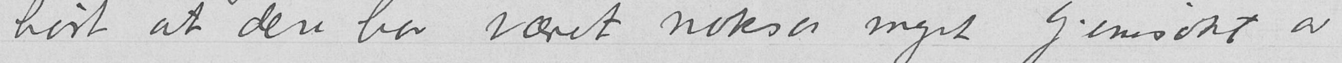hørt at dere har været noksaa meget hjemsøkt av
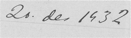20. des 1932
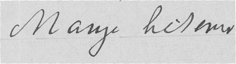Mange hilsener
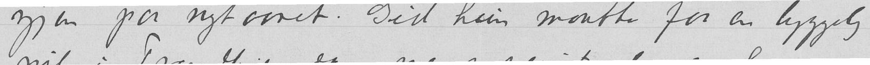igjen paa nytaaret. Gid hun maatte faa en hyggelig
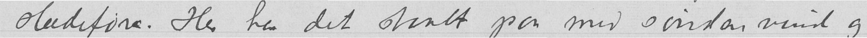sledeføre. Her har det staatt paa med søndenvind og
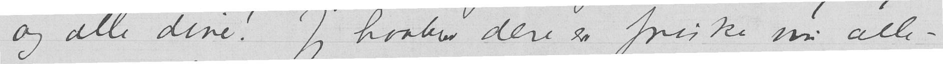og alle dine! Jeg haaber dere er friske nu alle-
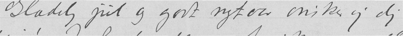Glædelig jul og godt nytaar ønsker jeg dig
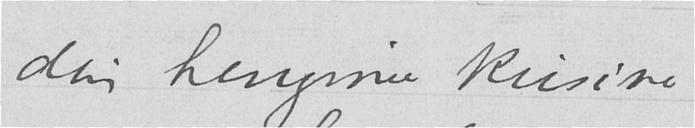din hengivne kusine
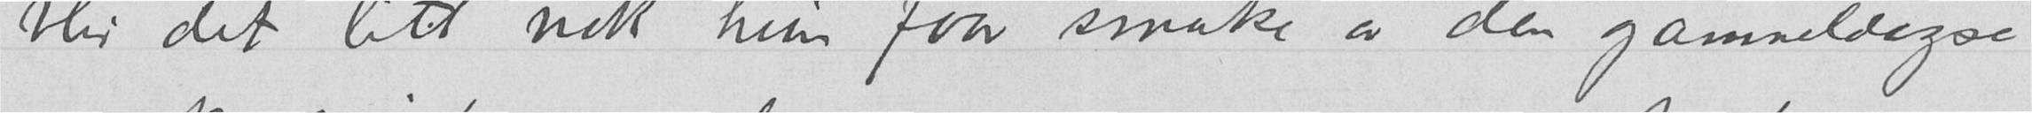blir det litt nok hun faar smake av den gammeldagse
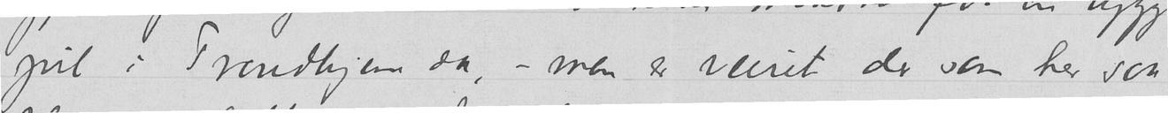jul i Trondhjem da, – men er veiret der som her saa
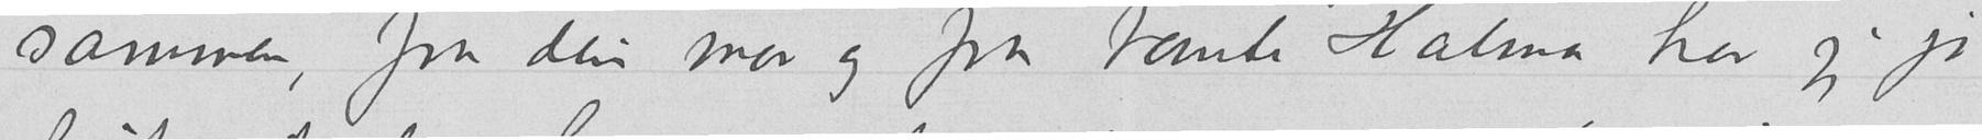sammen, fra din mor og fra tante Halma har jeg jo
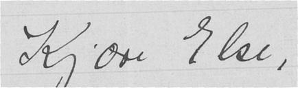Kjære Else,
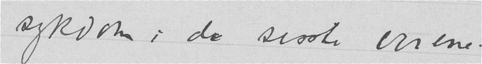sykdom i de sisste aarene.
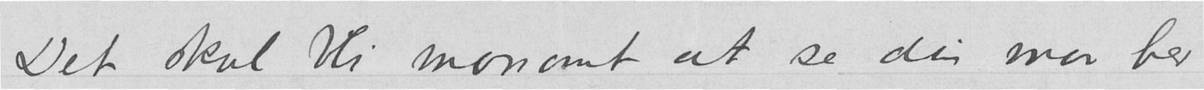Det skal bli morsomt at se din mor her
